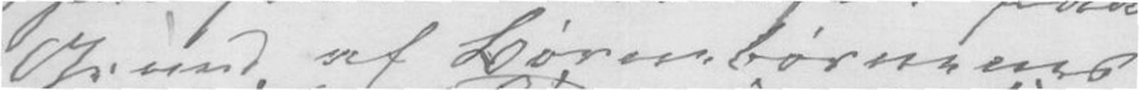Grund af Börnebörnenes
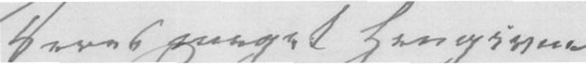Deres meget hengivne
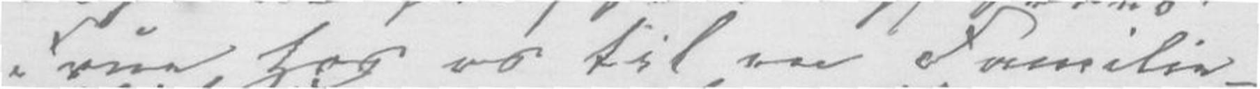Frue hos os til en Familie-
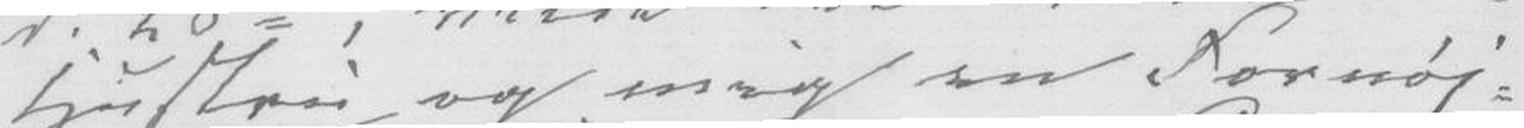Hustru og mig en Fornöj-
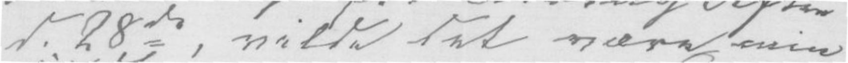d. 28de, vilde det være min
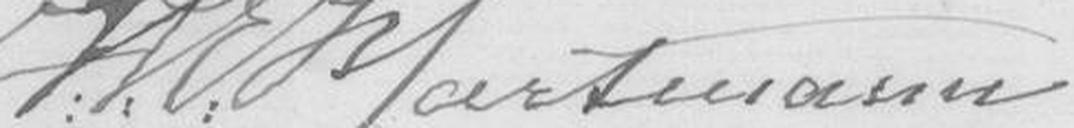J.P.E.Hartmann
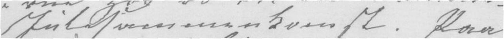Julesammenkomst. Paa
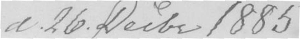d. 26. Decbr 1885.
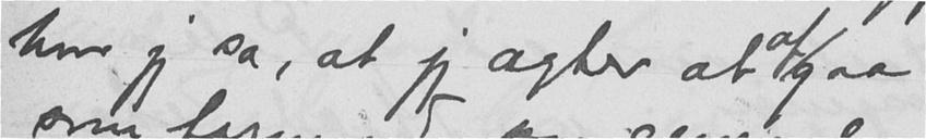hvor jeg sa, at jeg agter at gaa
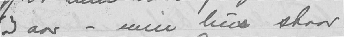3 aar - min hu staar
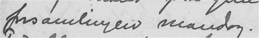forsamlingen mandag.
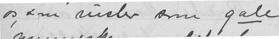os, som ruster som gale
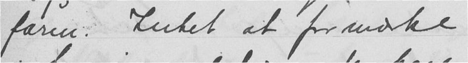form. Intet at formærke
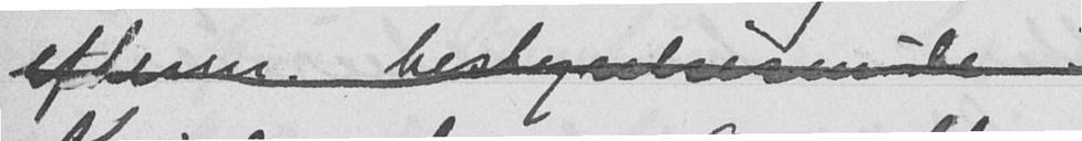efterm. bestyrelsesmøde i
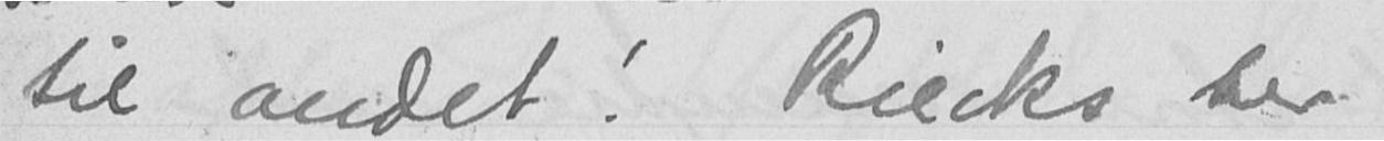til andet! Riecks her
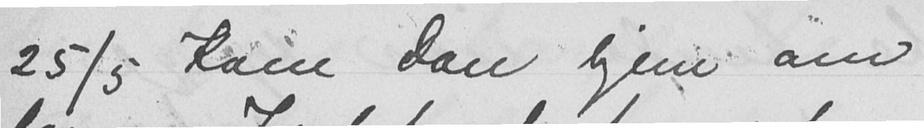25/5 Kom Don hjem om
